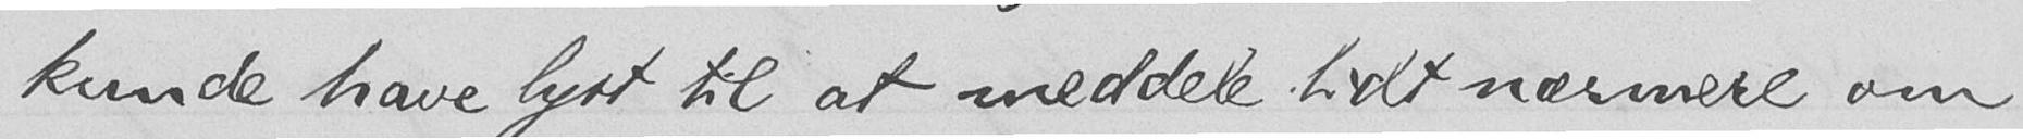kunde have lyst til at meddele lidt nærmere om
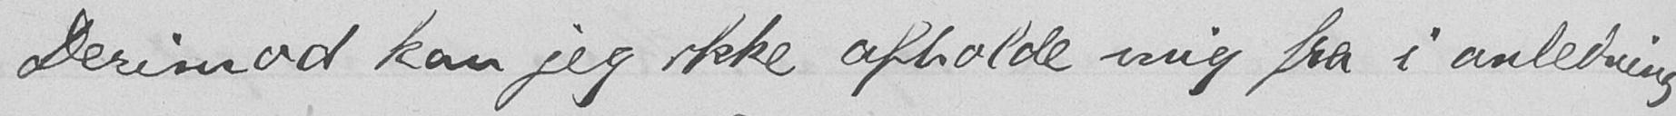Derimod kan jeg ikke afholde mig fra i anledning
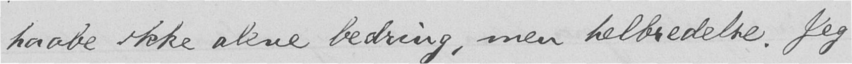haabe ikke alene bedring, men helbredelse. Jeg
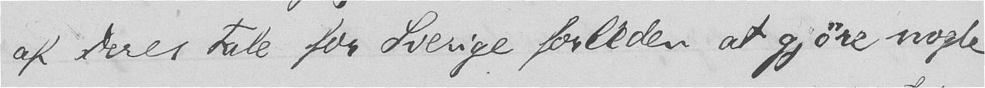af Deres tale for Sverige forleden at gjøre nogle
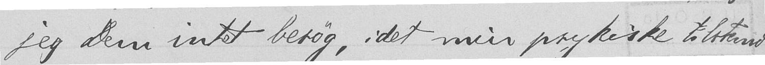jeg Dem intet besøg, idet min psykiske tilstand
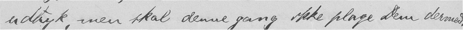udtryk, men skal denne gang ikke plage Dem dermed.
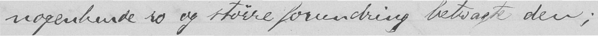nogenlunde ro og større forundring betragte den;
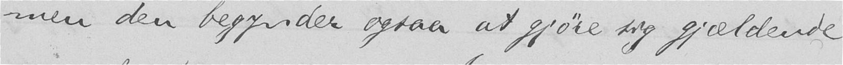men den begynder ogsaa at gjøre sig gjældende
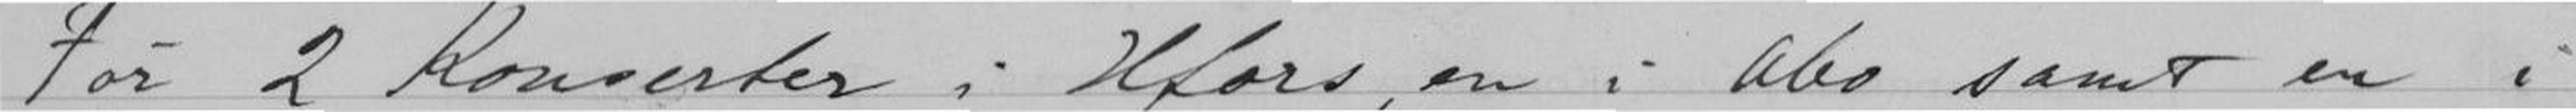För 2 Konserter i Hfors, en i Åbo samt en i
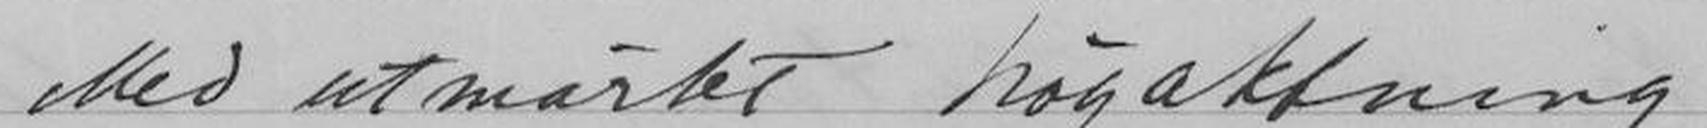Med utmärkt högaktning
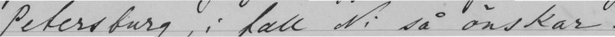Petersburg, i fall Ni så önskar.
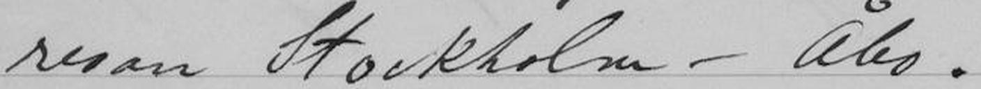resan Stockholm - Åbo.
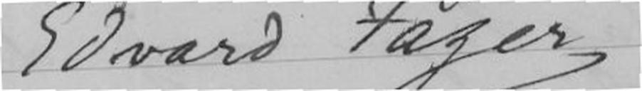Edvard Fazer
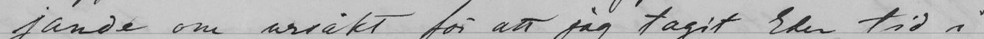jande om ursäkt för att jag tagit Eder tid i
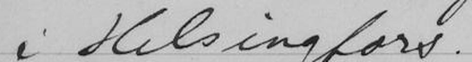i Helsingfors.
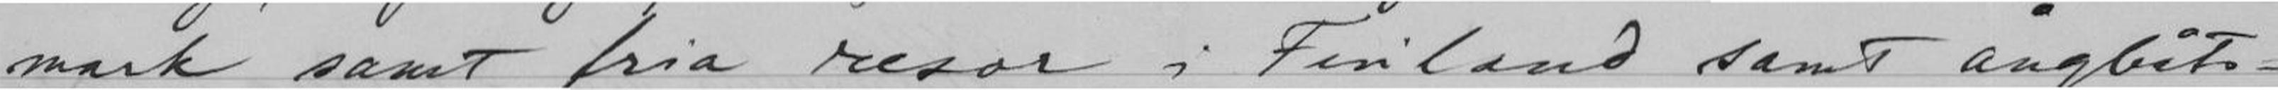mark samt fria resor i Finland samt ångbåts-
